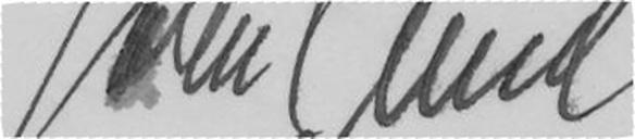John Lund
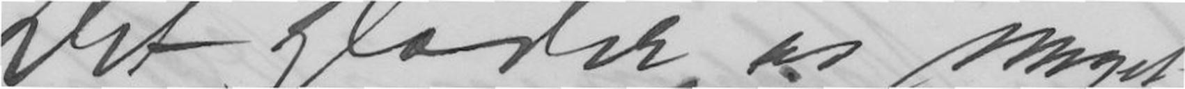Det glæder os meget
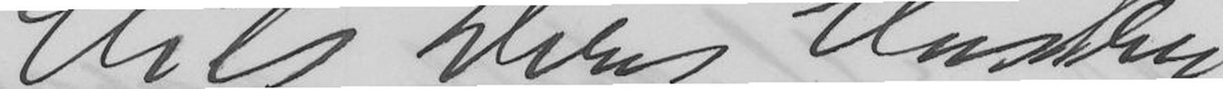Hils Deres Hustru
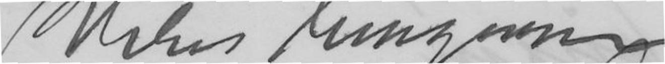Deres hengivne
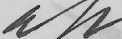op
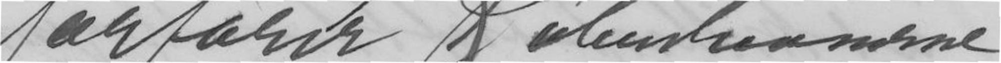forfører Københavnerne
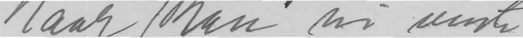Naar kan vi vente
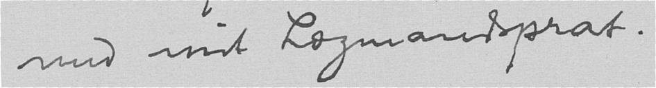med mit Lægmandsprat.
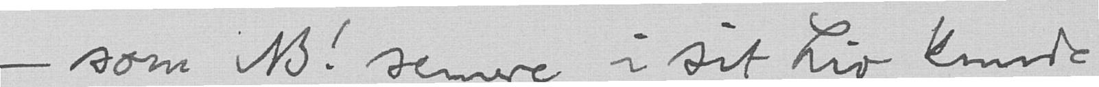- som NB! senere i sit Liv kunde
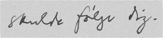skulde følge dig.
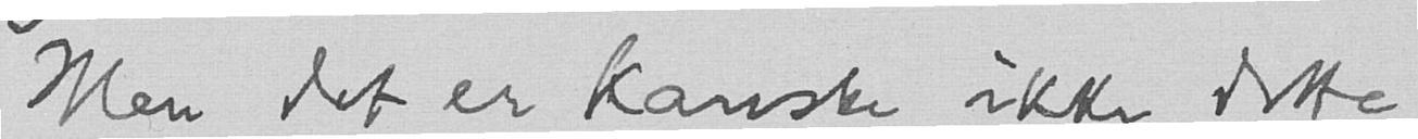Men det er kanske ikke dette
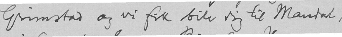Grimstad og vi fik bile dig til Mandal,
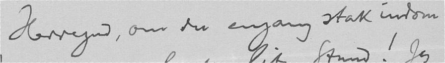Herregud, om du engang stak indom
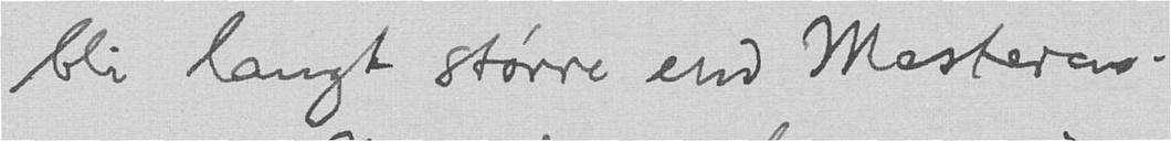bli langt større end Mesteren.
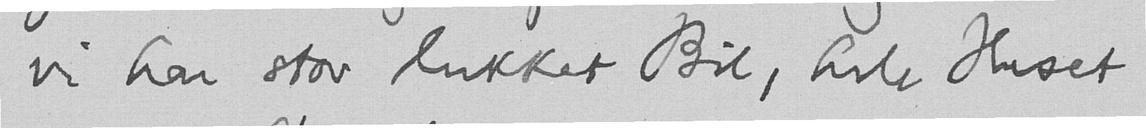vi har stor lukket Bil, hele Huset
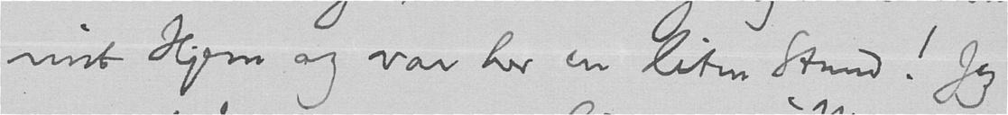mit Hjem og var her en liten Stund! Jeg
